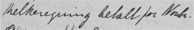Melkeregning betalt for Novbr.
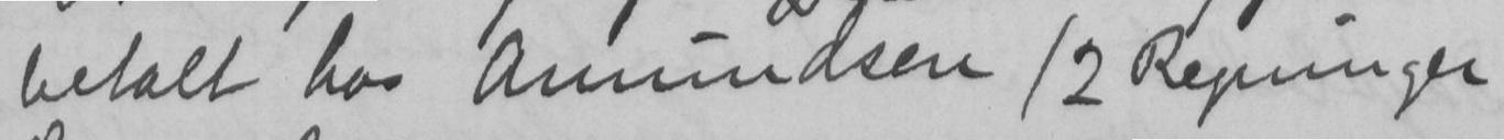betalt hos Amundsen / 2 Regninger
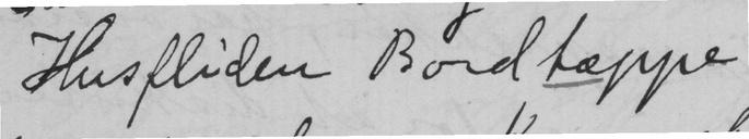Husfliden Bordtæppe
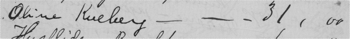Oline Kveberg - - - 31, 00
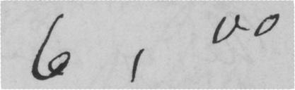6, 00
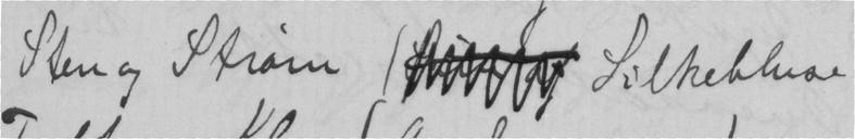Sten og Strøm / Silkebluse
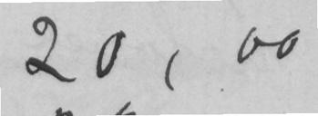20, 00
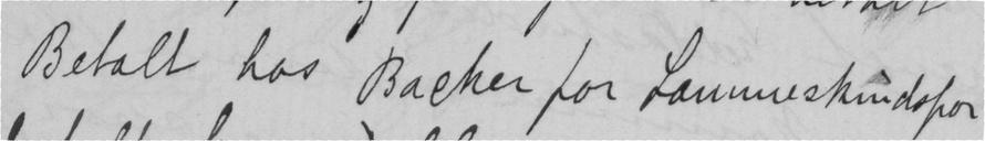Betalt hos Backer for Lammeskindsfor
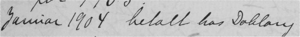Januar 1904 belalt hos Doblaug
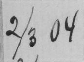2/3 04
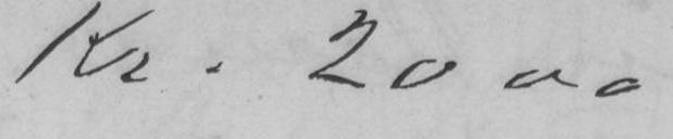Kr. 20 00
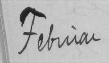Februar
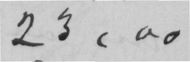23, 00
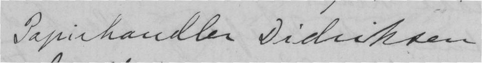Papirhandler Didriksen
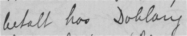betalt hos Doblaug
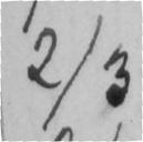2/3
